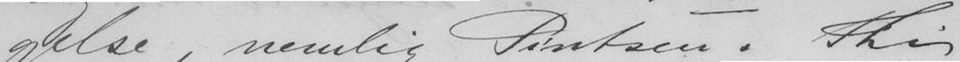gelse, nemlig Pintsen. Thi
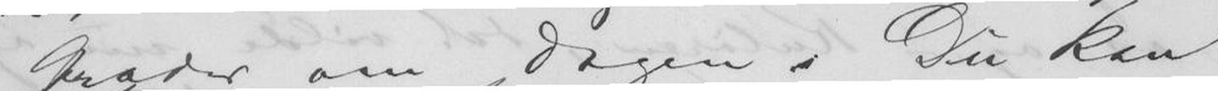Grader om Dagen. Du kan
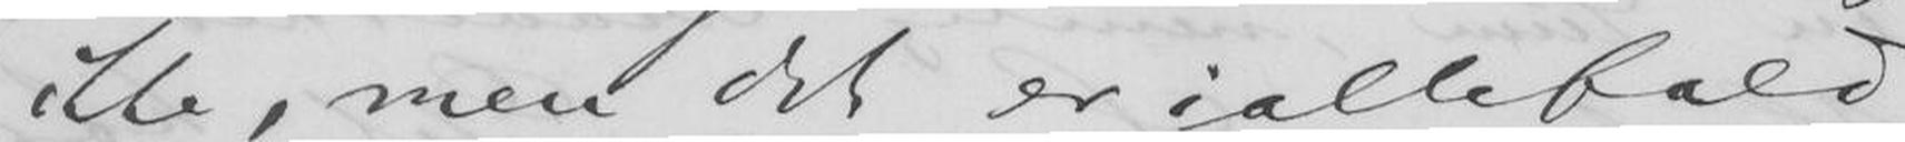ikke, men det er iallefald
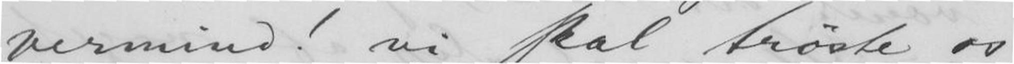vermind! vi skal trøste os,
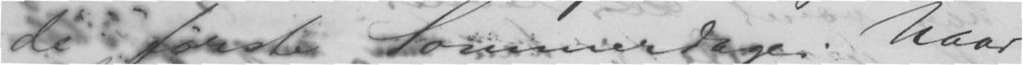de første Sommerdage.
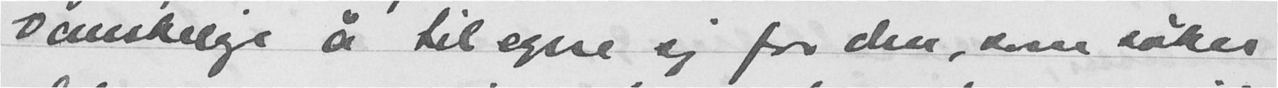vanskelige å tilegne sig for den, som søker
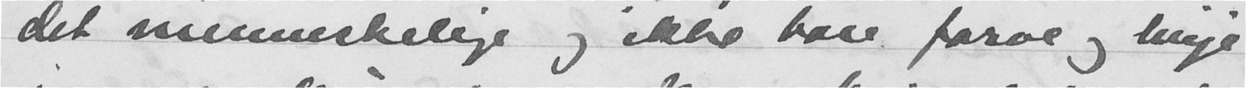det menneskelige og ikke bare farve og linje
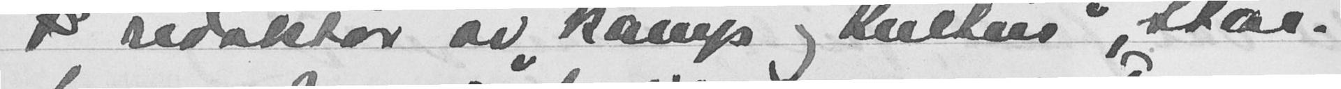B redaktør av "Kamp og Kultur", Stai.
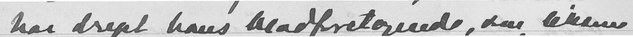har drept hans bladforetagende, som, liksom
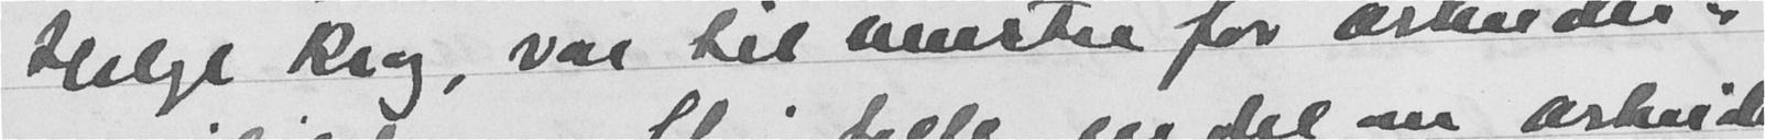Helge Krog, var til venstre for arbeider-
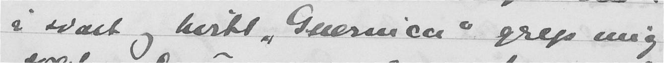i svart og hvitt "Guernica" grep mig
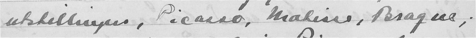utstillingen, Picasso, Matisse, Braque,
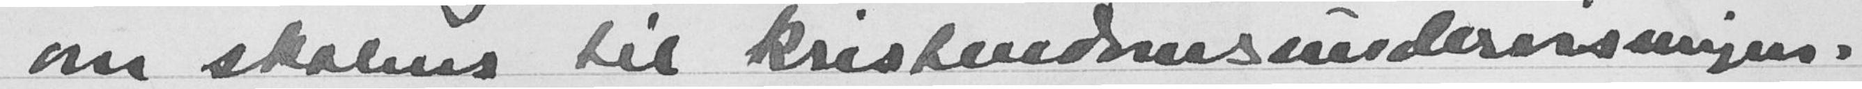om skolens til kristendomsundervisningen.
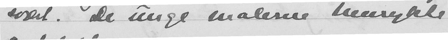svært. De unge malerne henrykte
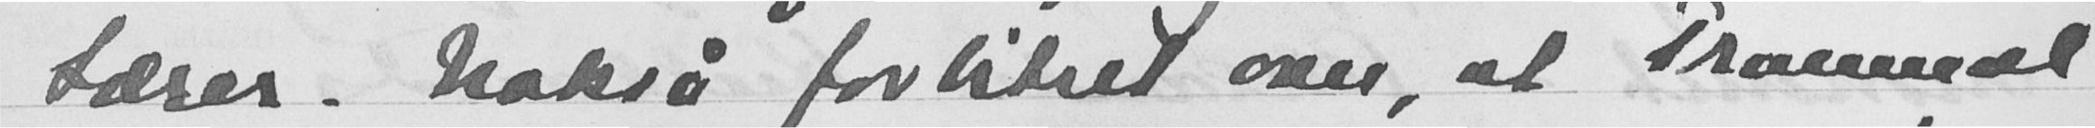Lærer. Nokså forbitret over, at Tranmæl
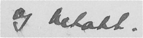og betatt.
